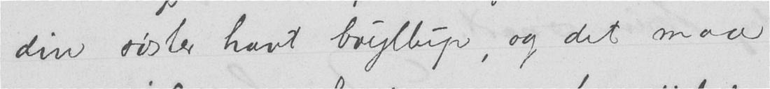din søster havt bryllup, og det maa
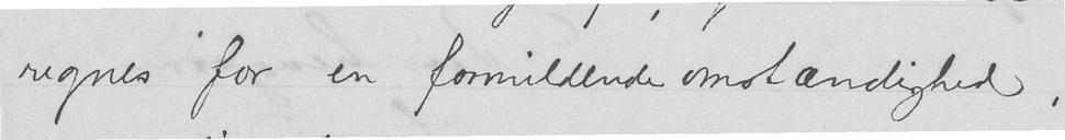regnes for en formildende omstændighed,
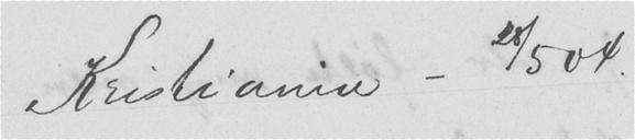Kristiania - 28/5 04.
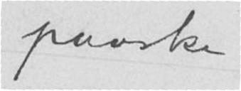paaske - .
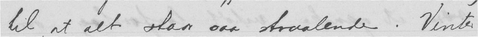til, at alt staar saa straalende. Vinter
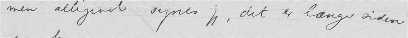men alligevel synes jeg, det er længe siden
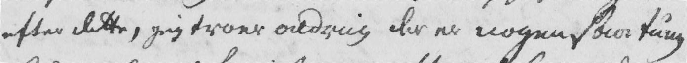efter dette, jeg troer aldrig der er nogen saa tung-
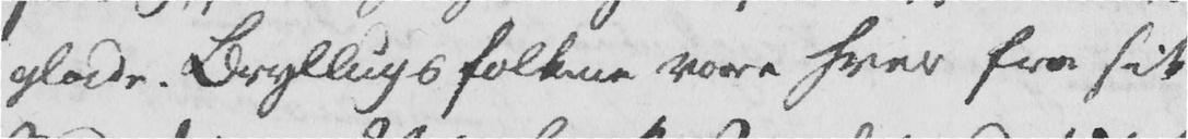glade. Bryllupsfolkene vare hver fra sit
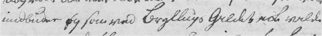indbudne og som ved Bryllups Gildet icke vilde
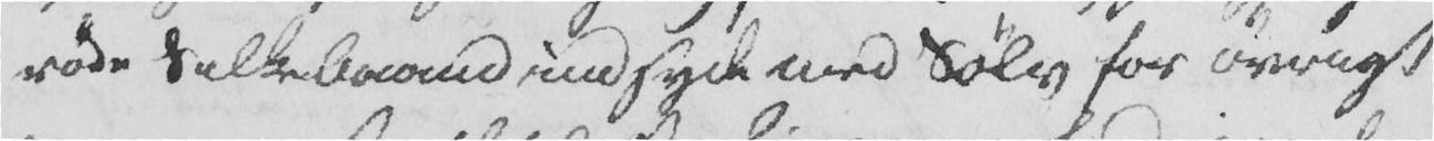røde Silkebaand indsyde med Sølv for øvrigt
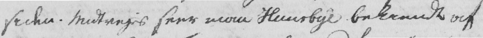siden. Midtvejes seer man Huusbye bekiendt af
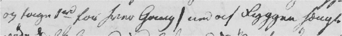og tage 1 rd for hver Gang :/ ned af Ryggen hængte
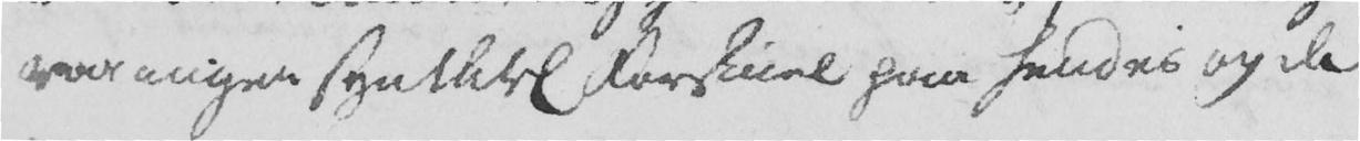var ingen synderl Forskiel paa hendes og de
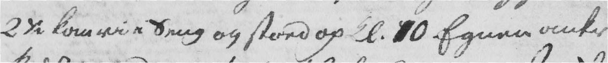2 1/2 kom vi i Seng og stoed op kl. 10. Egnen omkr
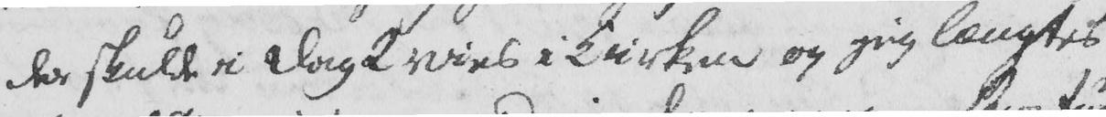der skulde i Dag 2 vies i Kirken og jeg længtes
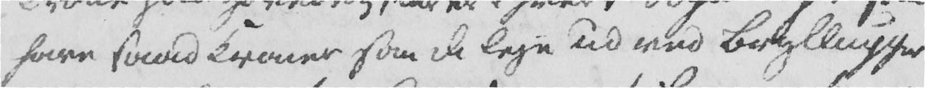have saad Kroner som de leje ud ved Bryllupper
